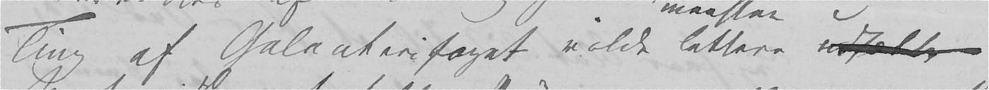Ting af Galanterifaget vilde maaskee lettere udsætte
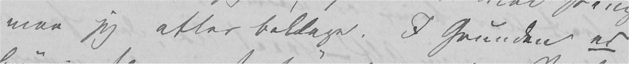maa jeg atter beklage. I Grunden er
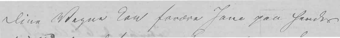Dine Vegne kan forære Jane paa hendes
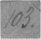103.
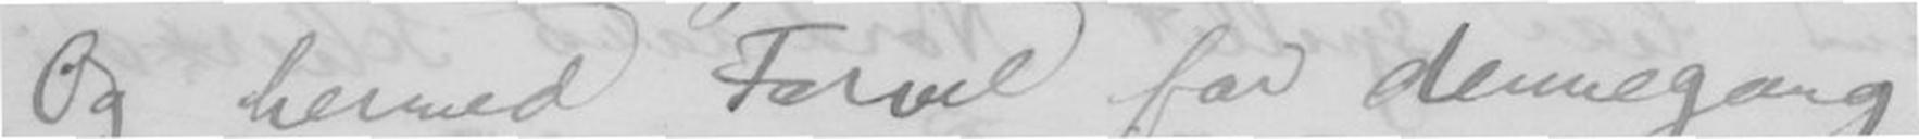Og hermed Farvel for denne gang,
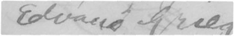Edvard Grieg
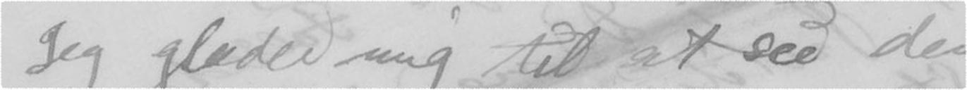Jeg glæder mig til at see den
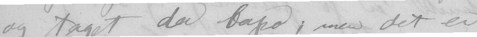og taget da Capo; men det er
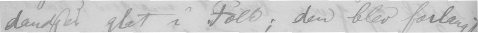dandsen glat i Folk; den blev forlangt
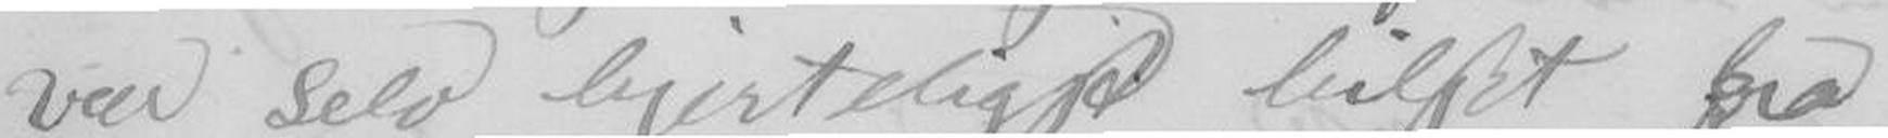vær selv hjerteligst hilset fra
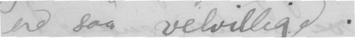ere saa velvillige.
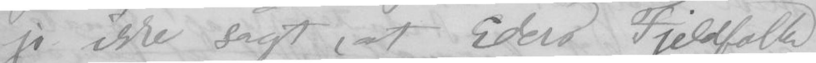jo ikke sagt; at Eders Fjelfolk
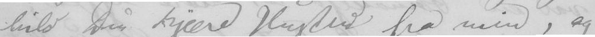hils Din kjære Hustru fra min, og
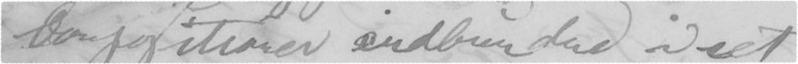Compositurer inbundne i et
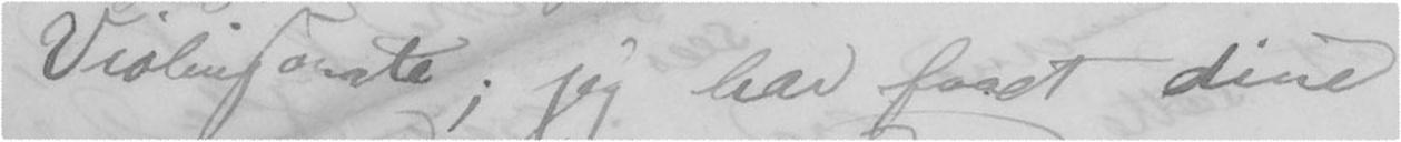Violinsonate; jeg har faaet dine
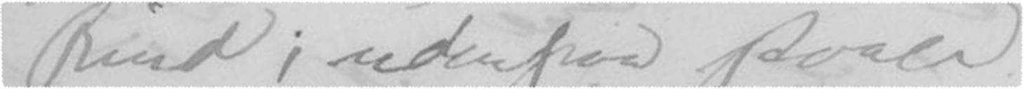Bind; udenpaa staaer
